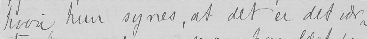hvori hun synes, at det er det vær-
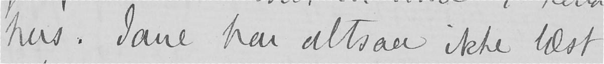hus. Jane har altsaa ikke læst
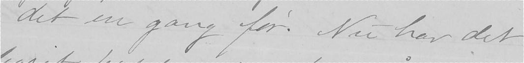det en gang før. Nu har det
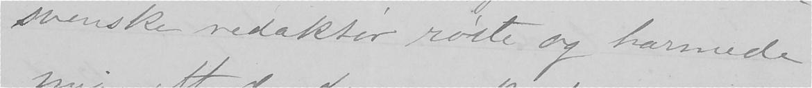svenske redaktør rørte og harmede
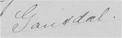Gausdal.
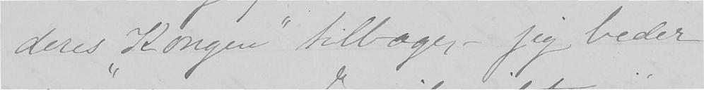deres "Kongen" tilbage, - jeg beder
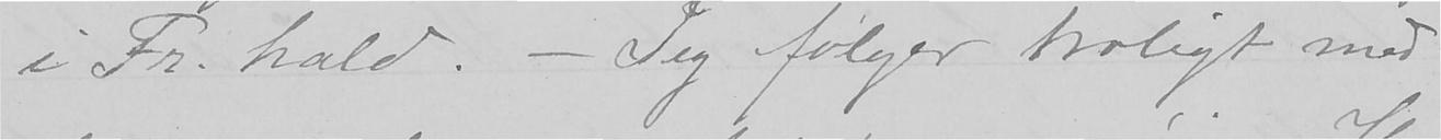i Fr. hald. - Jeg følger troligt med
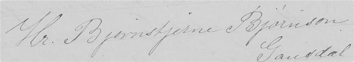Hr. Bjørnstjerne Bjørnson
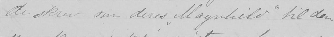de skrev om deres "Magnhild" til den
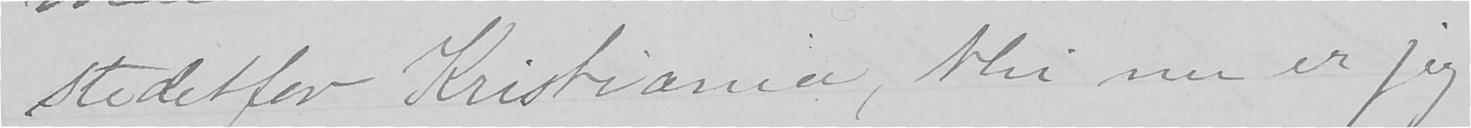stedetfor Kristiania, thi nu er jeg
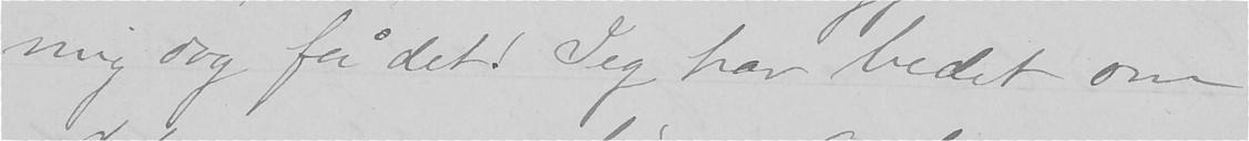mig dog få det! Jeg har bedet om
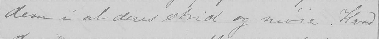dem i al deres strid og møie. Hvad
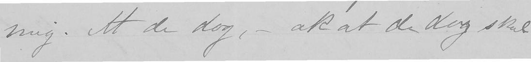mig. At de dog, - ak at de dog skal
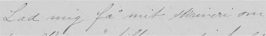Lad mig få mit skriveri om
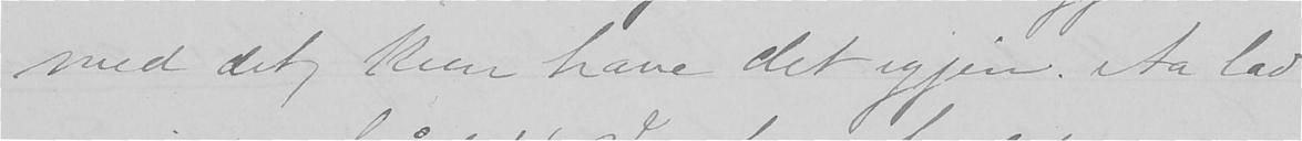med det, kun have det igjen. Aa lad
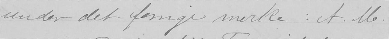under det forrige merke: A.M.
